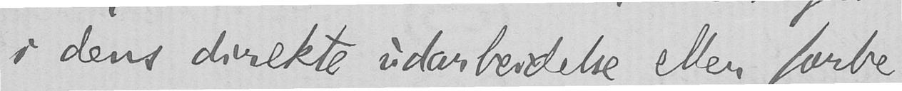i dens direkte udarbeidelse eller forbe-
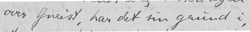over Gneist, har det sin grund i,
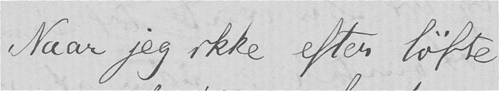Naar jeg ikke efter løfte
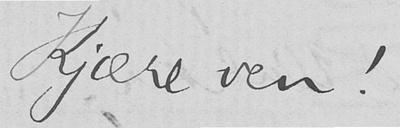Kjære ven!
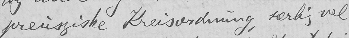preussiske Kreisordnung, særlig vel
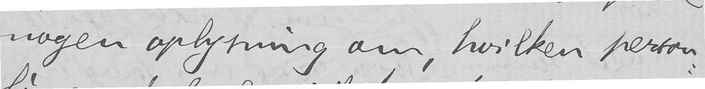nogen oplysning om, hvilken person-
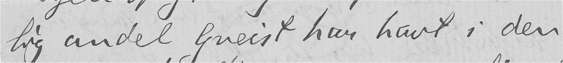lig andel Gneist har havt i den
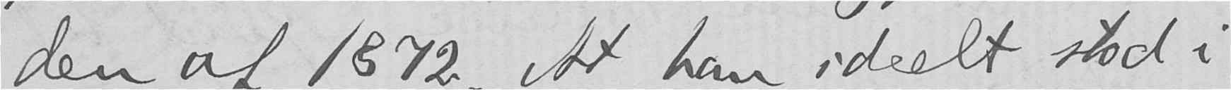den af 1872. At han ideelt stod i
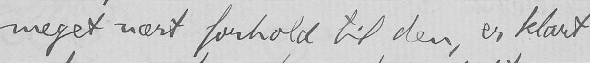meget nært forhold til den, er klart
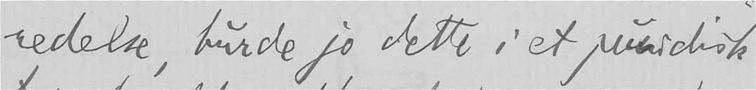redelse, burde jo dette i et juridisk
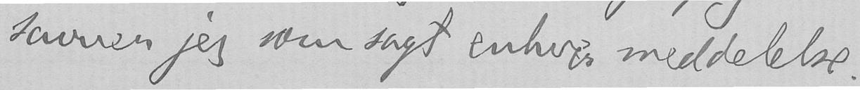savner jeg som sagt enhver meddelelse.
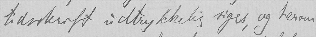tidsskrift udtrykkelig siges, og herom
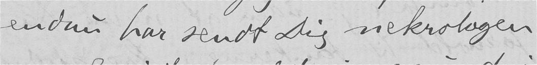endnu har sendt Dig nekrologen
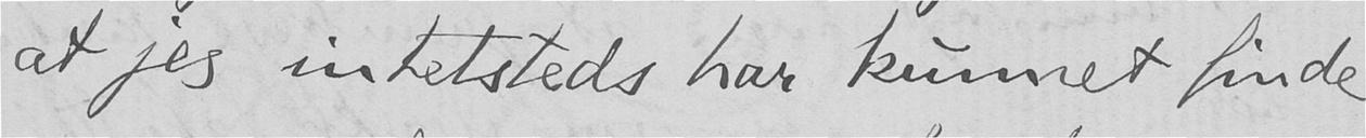at jeg intetsteds har kunnet finde
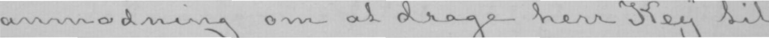anmodning om at drage herr Key til
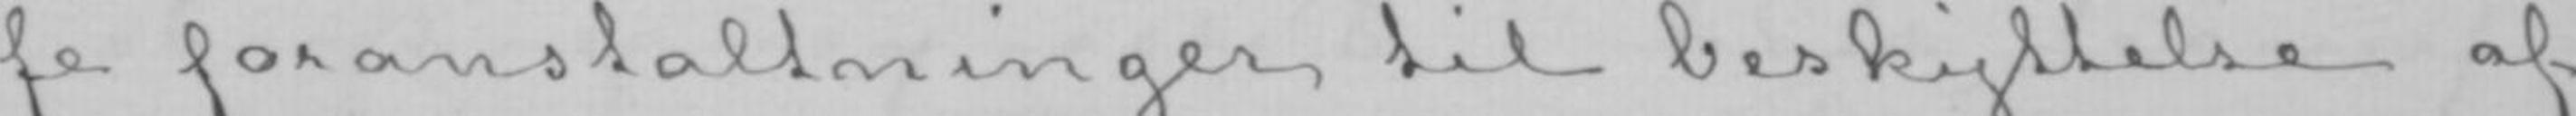fe foranstaltninger til beskyttelse af
